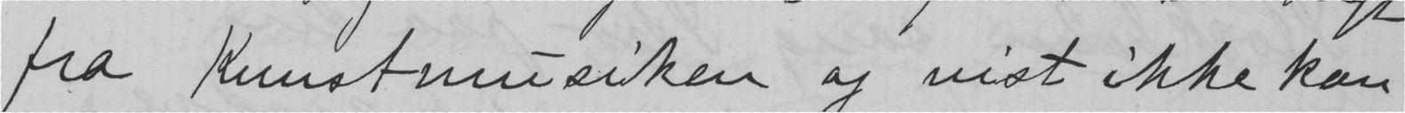fra Kunstmusiken og vist ikke kan
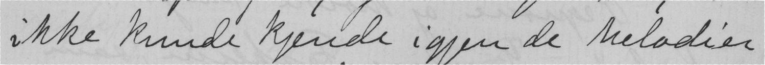ikke kunde kjende igjen de melodier
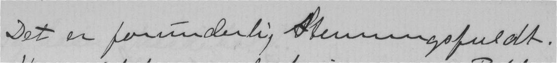Det er forunderlig stemningsfuldt.
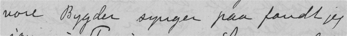vore Bygder synger paa fandt jeg
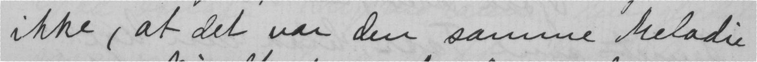ikke, at det var den samme Melodie
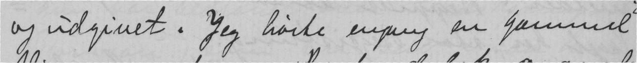og udgivet. Jeg hørte engang en gammel
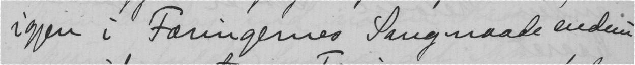igjen i Færingernes Sangmaade endnu
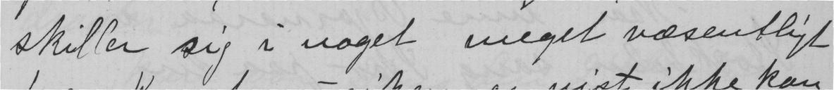skiller sig i noget meget væsentligt
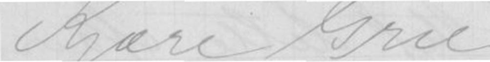Kjære Grieg!
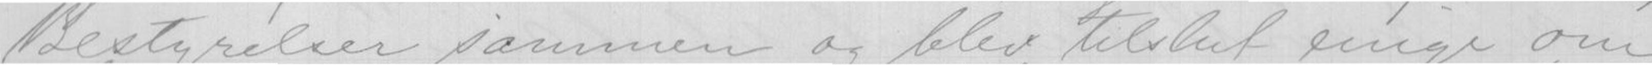BestyrelseR sammen og blev tilslut enige om
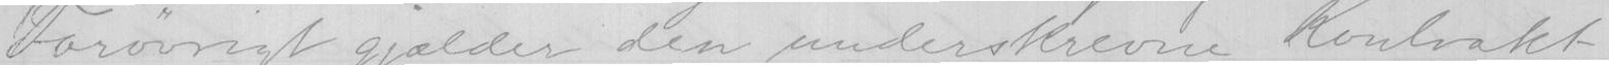Forøvrigt gjælder den underskrevne Kontrakt
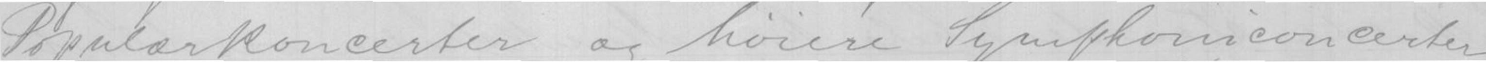Populærkoncerter og høiere Symphoniconcerter
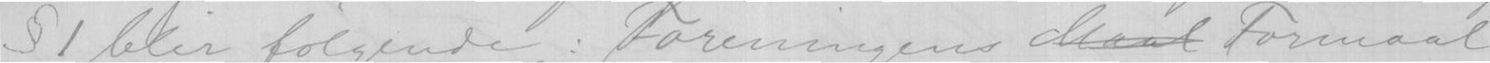§1 blir følgende: Foreningens Maal Formaal
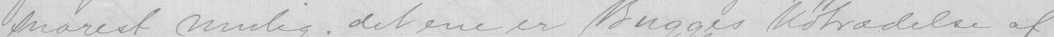snarest mulig; det ene er Bugges Udtrædelse af
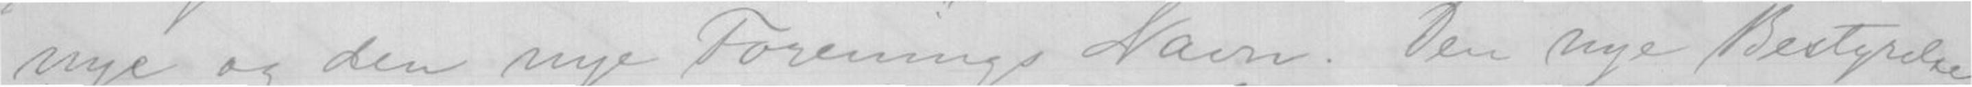nye, og den nye Forenings Navn. Den nye Bestyrelse
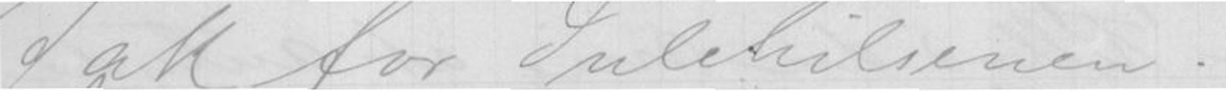Tak for Julehilsenen!
